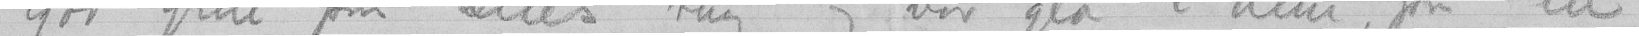god greie paa Deres ting og var gla i dem, paa en
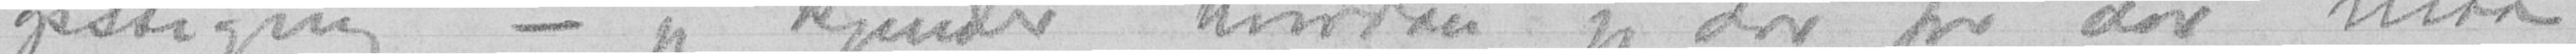opstigning - jeg kjender hvordan jeg aar for aar maa
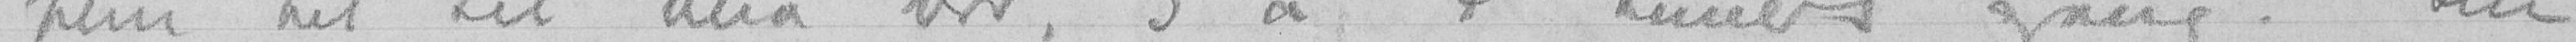frem hit til bua vor, 3 a 4 timers gang. Her
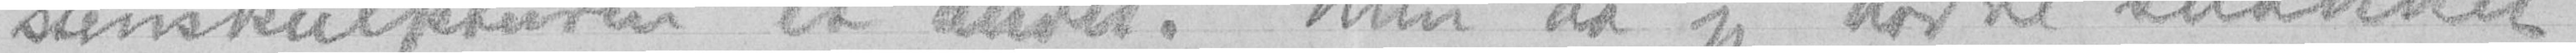stenskulpturen et andet. Men da jeg hadde snakket
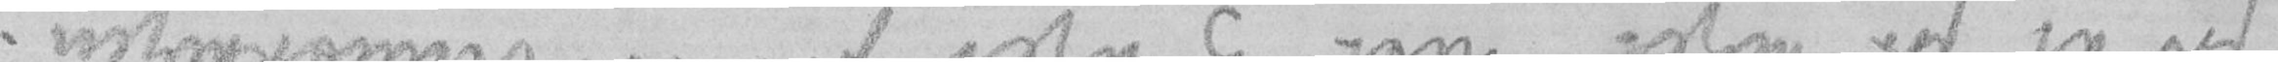for at faa noget hist og noget paa St. Hanshaugen.
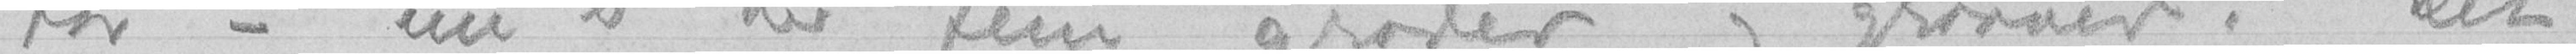tør - nu er her fem grader og graaveir. Det
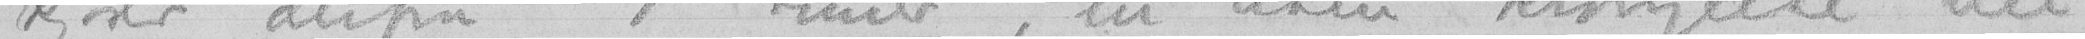kjører derfra 4 timer, en liten kronglete vei
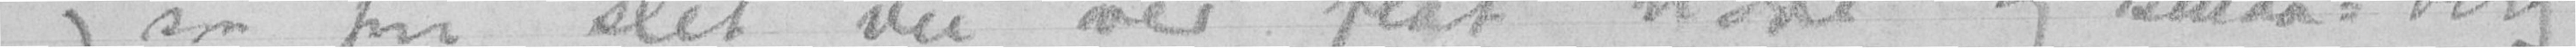og saa fin slet vei over flat vidde og smaa-berg
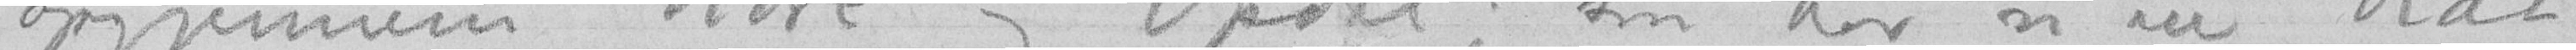opgjennem Nore og Opdal; saa har vi en brat
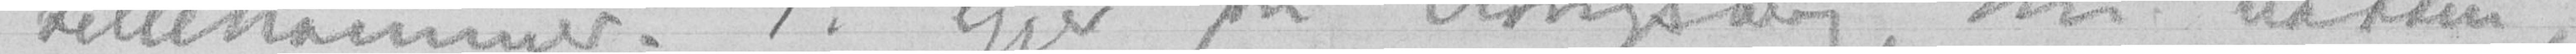Lillehammer. Vi ligger paa Kongsberg, om natten,
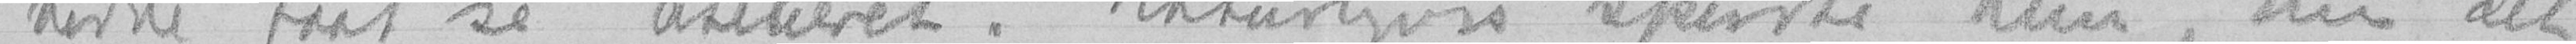hadde faat se atelieret. Naturligvis spurte hun, om det
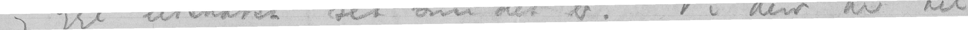og ligge utstrakt ret som det er. Vi blir her til
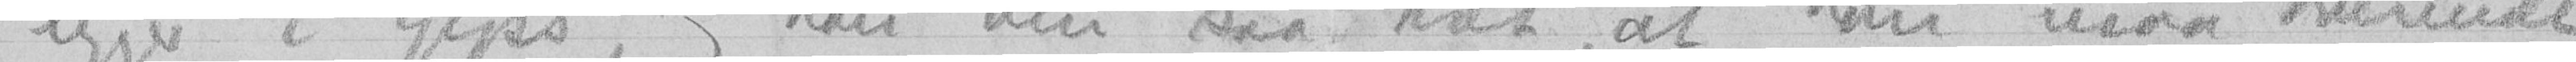ligger i gips, og han blir saa træt, at han maa overende
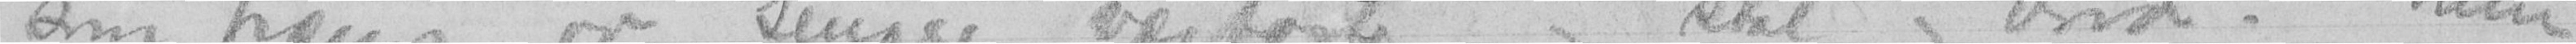som trængs av senger, vægfaste, og stor og bord. Men
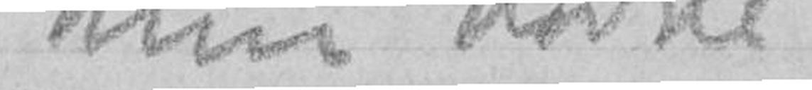hun hadde
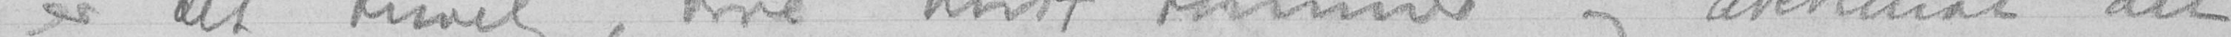er det trivelig, bare hvitt tømmer og akkurat det
